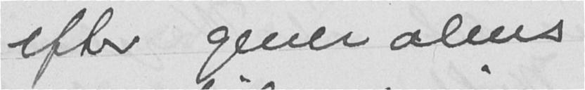efter generalens
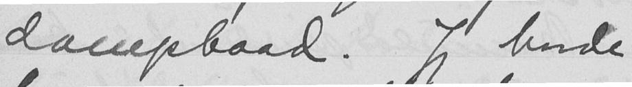dampbaad. Jeg havde
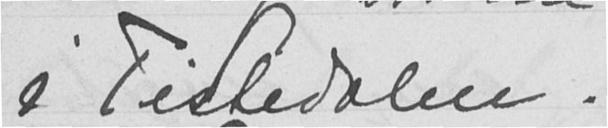i Tistedalen.
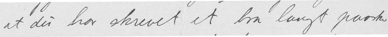at du har skrevet et bra langt paaske-
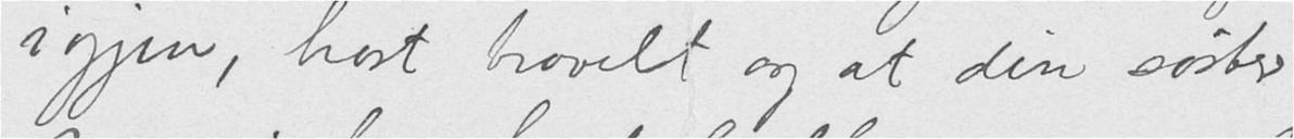igjen, havt travelt og at din søster
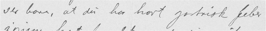ser bare, at du har havt gastrisk feber
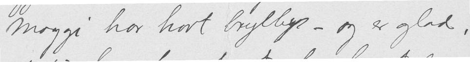Maggi har havt bryllup - og er glad,
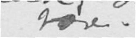være.
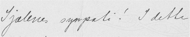Sjælenes sympati! I dette
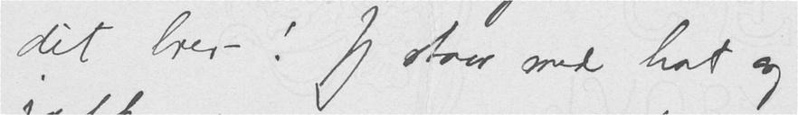dit brev! Jeg staar med hat og
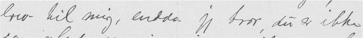brev til mig, endda jeg tror, du er ikke
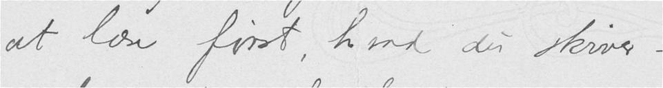at læse først, hvad du skriver -
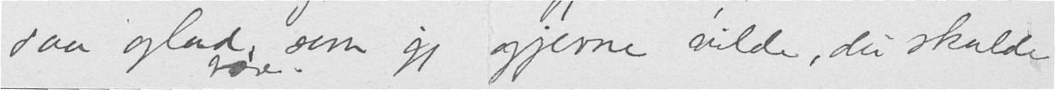saa glad, som jeg gjerne vilde, du skulde
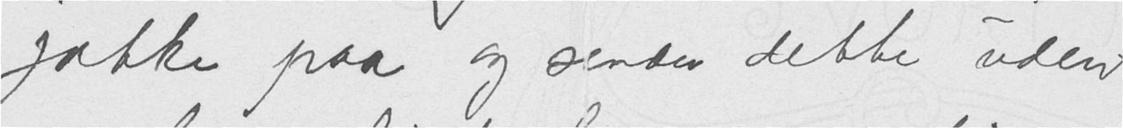jakke paa og sender dette uden
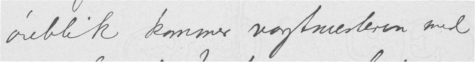øieblik kommer vagtmesteren med
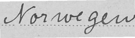Norwegen
